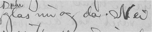glas nu og da. Nei
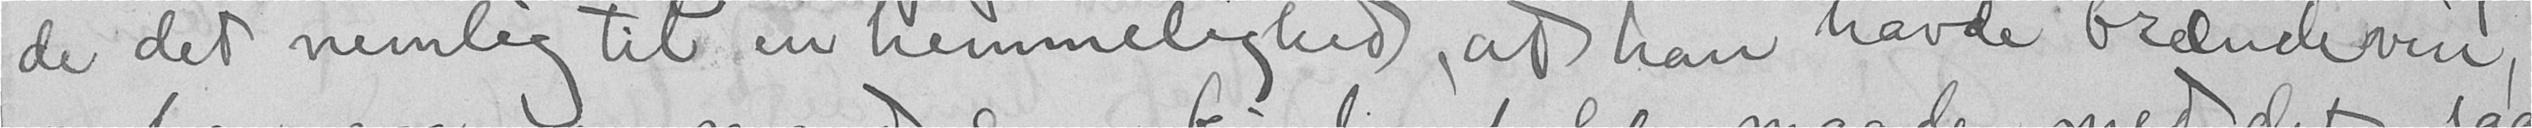de det nemlig til en hemmelighed, at han havde brændevin,
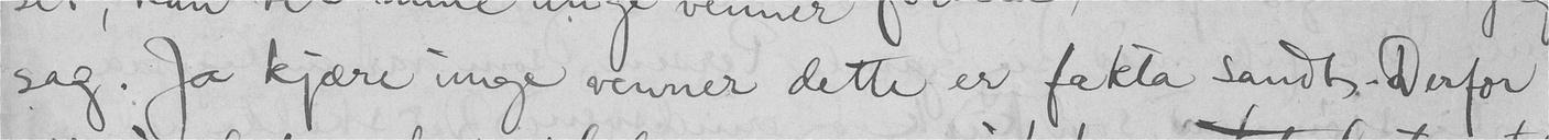sag. Ja kjære unge venner dette er fakta sandt. Derfor
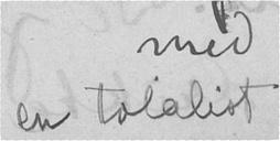en totalist
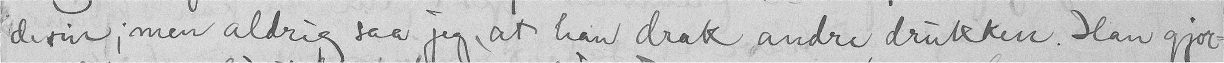devin; men aldrig saa jeg, at han drak andre drukken. Han gjor-
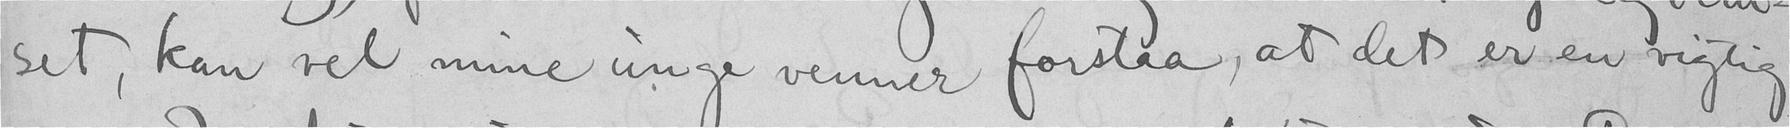set, kan vel mine unge venner forstaa, at det er en rigtig
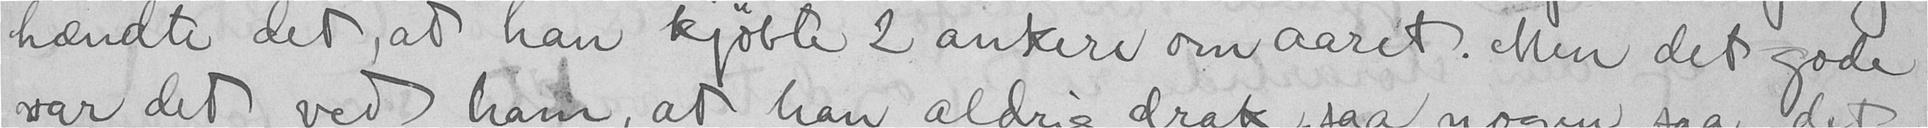hændte det, at han kjøbte 2 ankere om aaret. Men det gode
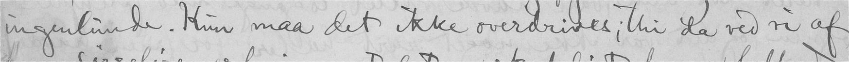ingenlunde. Kun maa det ikke overdrives; thi da ved vi af
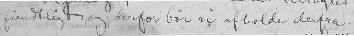fiendtligt, og derfor bør vi afholde derfra.
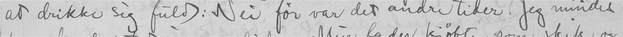at drikke sig fuld: Nei før var det andre tider. Jeg mindes
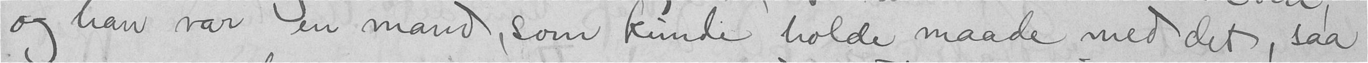og han var en mand, som kunde holde maade med det, saa
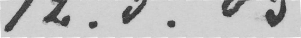12.5.03.
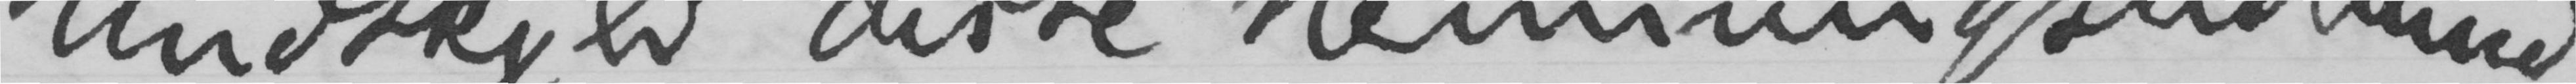Undskyld disse stemningsudbrud,
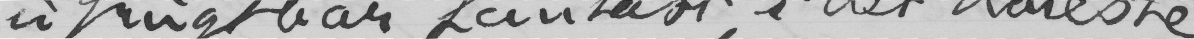ufrugtbar fantast, i det høieste
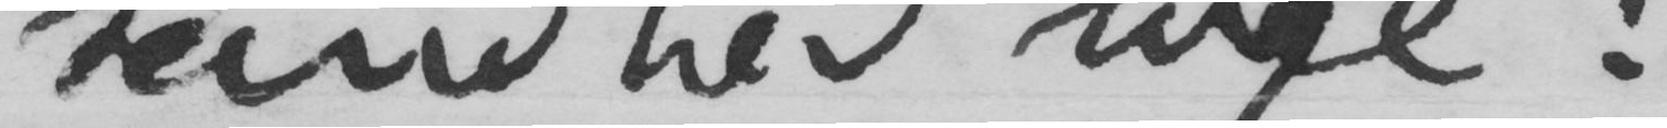sandhed sige:
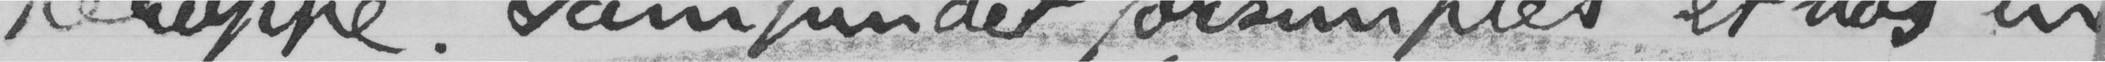heroppe. Samfundet forsimples et nos in
